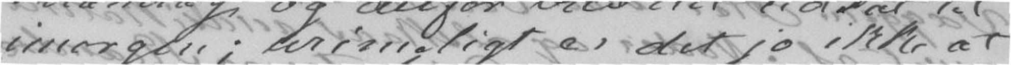imorgen; urimeligt er det jo ikke at
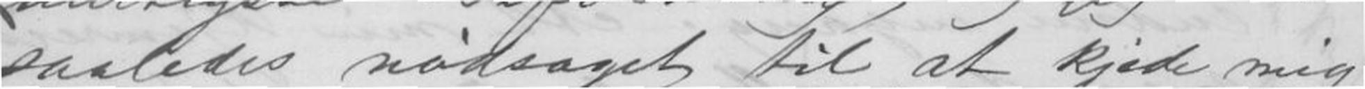saaledes nødsaget til at kjede mig
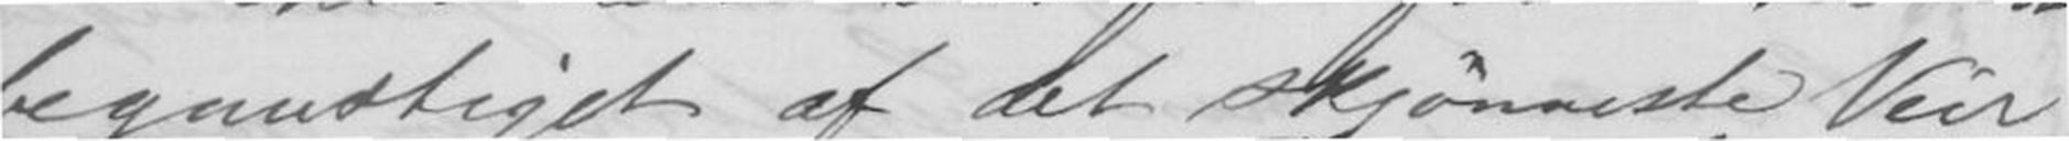begunstiget af det skjønneste Veir,
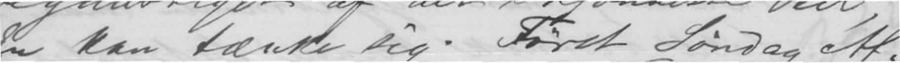En kan tænke sig. Først Søndag Af-
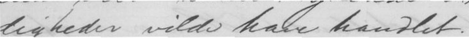digheder vilde have handlet.
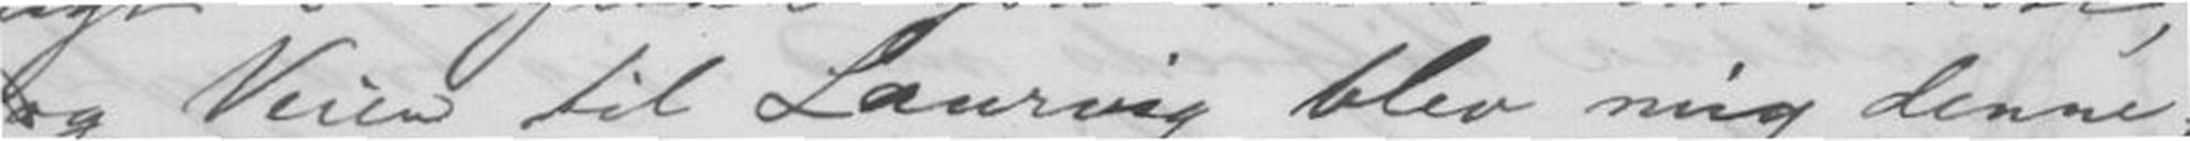og Veien til Laurvig blev mig denne
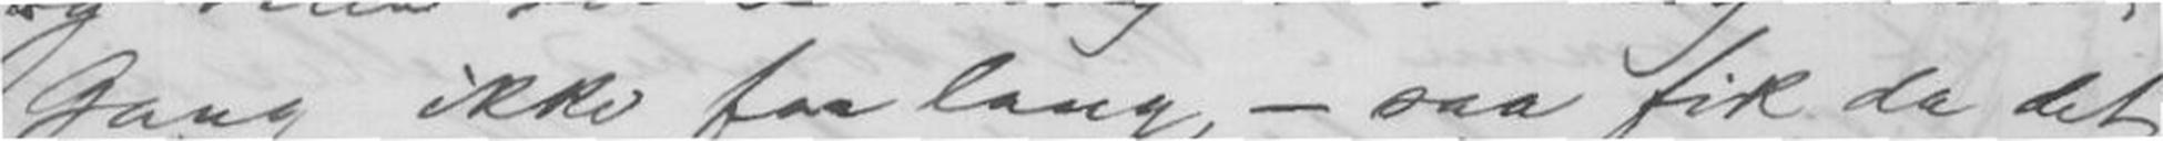Gang ikke for lang, - saa fik da det
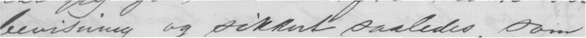bevisning og sikkert saaledes, som
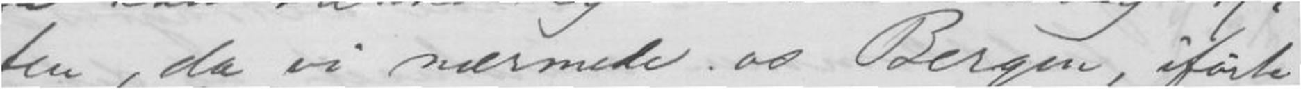ten, da vi nærmede os Bergen, iførte
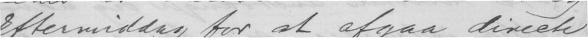Eftermiddag for at afgaa directe
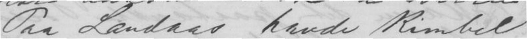Paa Landaas havde Kimbel
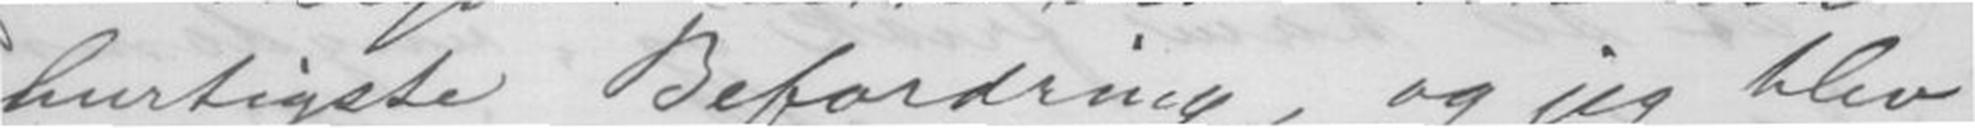hurtigste Befordring, og jeg blev
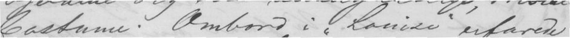Costume. Ombord i Louise erfarede
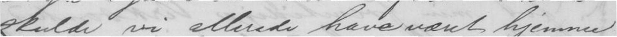skulde vi allerede have været hjemme
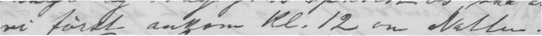vi først ankom Kl.12 om Natten.
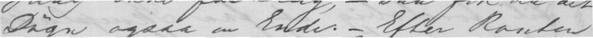Døgn ogsaa en Ende. - Efter Routen
